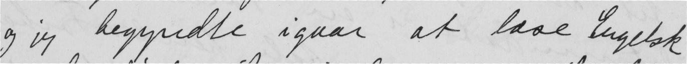og jeg begyndte igaar at læse Engelsk
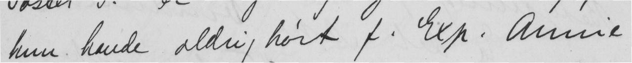hun havde aldrig hørt f. Exp. Annie
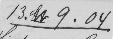13. 9.04
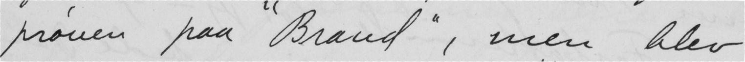prøven paa "Brand", men blev
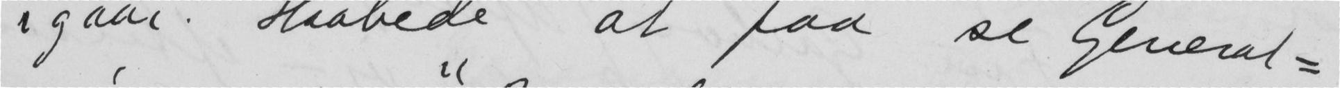igaar. Haabede at faa se General-
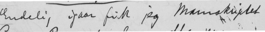Endelig igaar fik jeg Manuskriptet
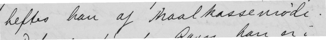heftes han af Maalkassemøde.
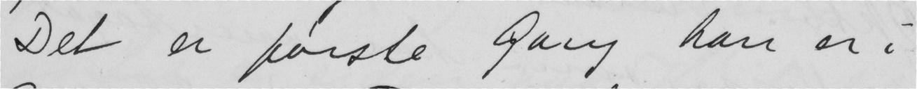Det er første Gang han er i
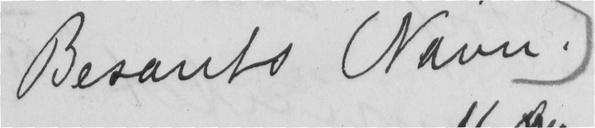Besants Navn.
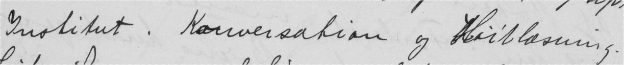Institut. Konversation og Høitlæsning.
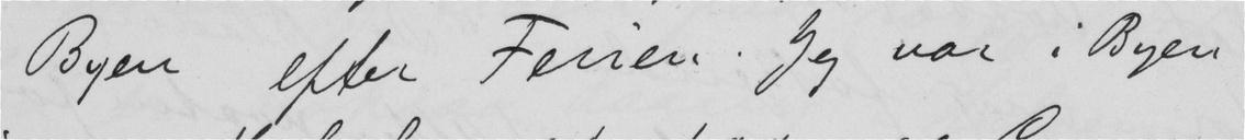Byen efter Ferien. Jeg var i Byen
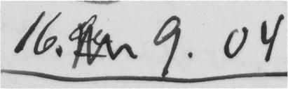16. 9.04
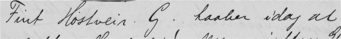Fint Høstveir. G. haaber idag at
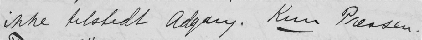ikke tilstedt Adgang. Kun Pressen.
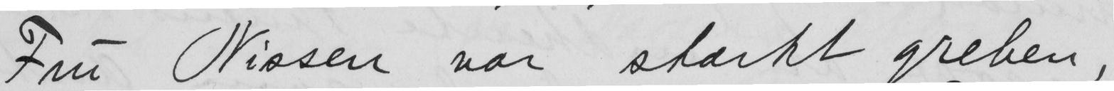Fru Nissen var stærkt greben,
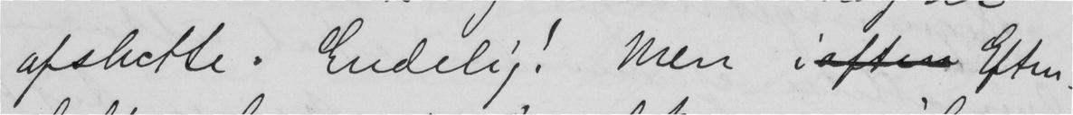afslutte. Endelig! Men i Eften.
